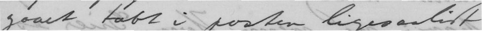gaaet tabt i posten, ligesaalidt
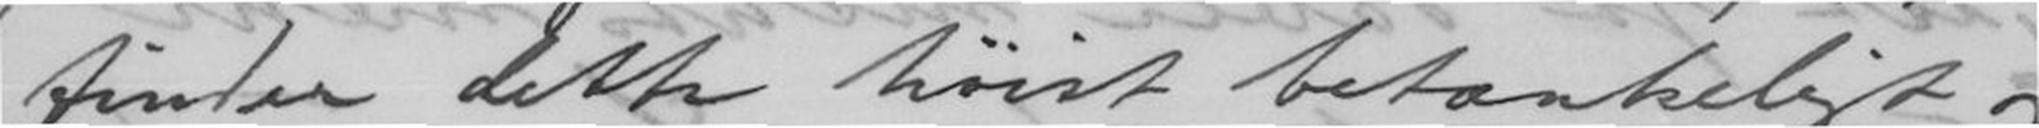finder dette høist betænkeligt og
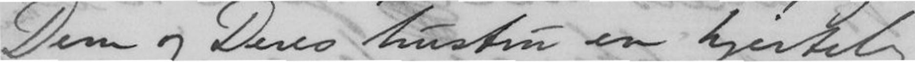Dem og Deres hustru en hjertelig
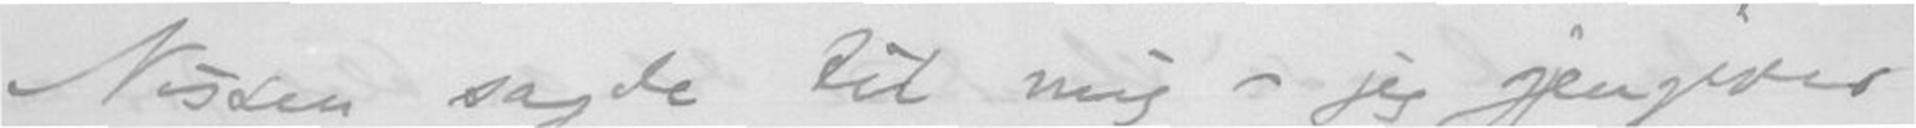Nissen sagde til mig - jeg gjengiver
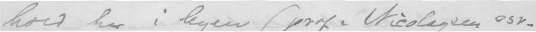hold her i byen (prof. Nicolajsen osv.
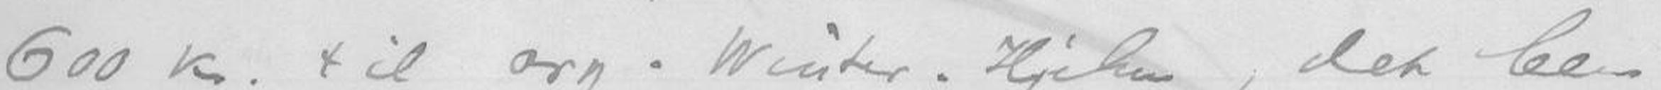600 kr. til org. Winter-Hjelm, det be-
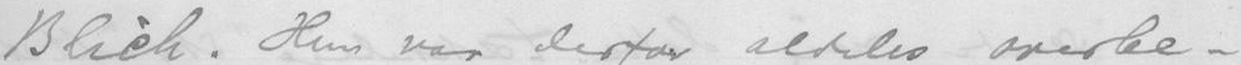Blich. Hun var derfor aldeles overbe-
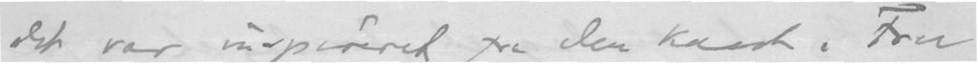det var inspireret fra den kant. Fru
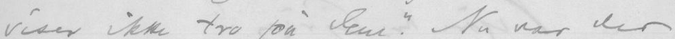viser ikke tro på Dem". Nu var der
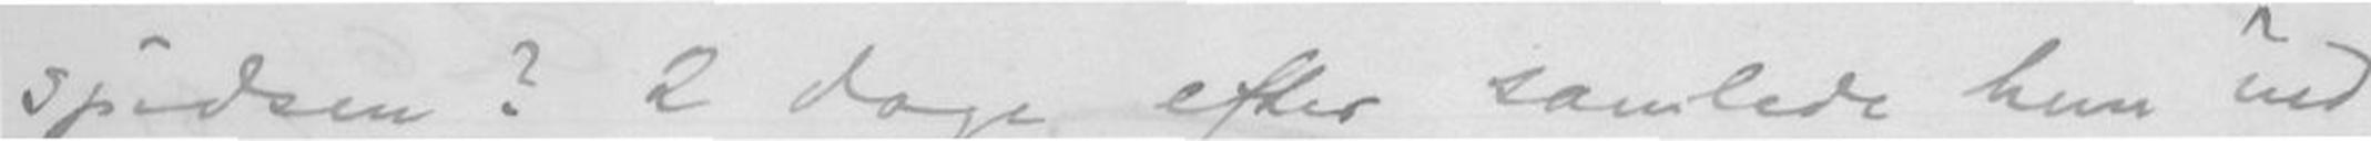spidsen? 2 dage efter samlede hun ind
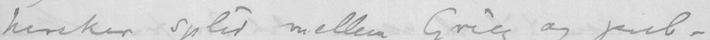hersker spild mellem Grieg og pub-
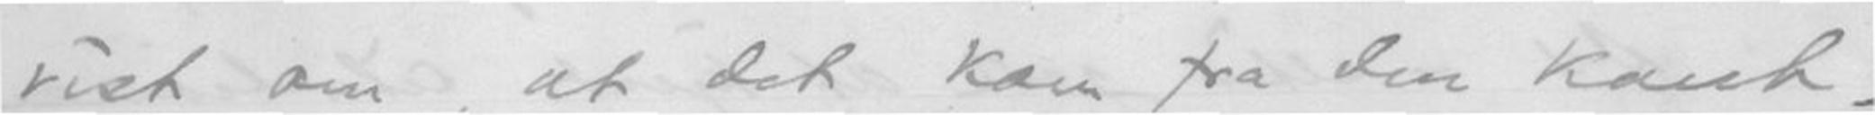vist om, at det kom fra den kant -
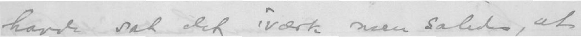havde sat det iværk men således, at
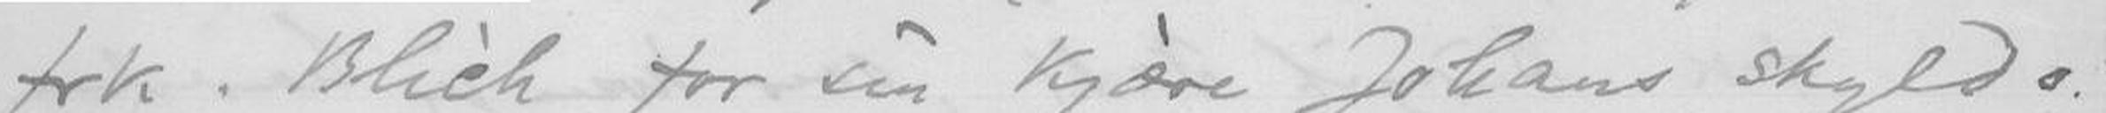frk. Blich for sin kjære Johans skyld o.
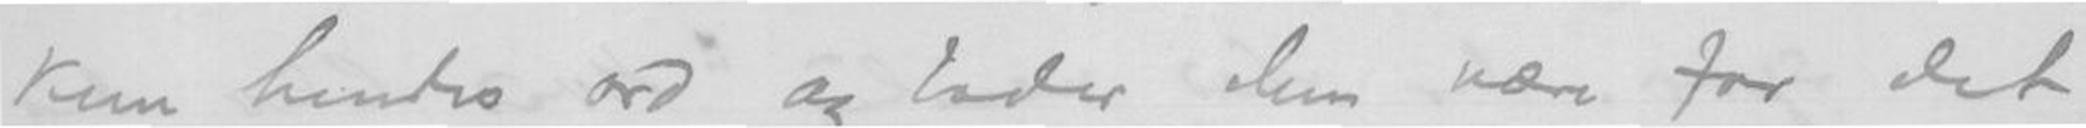kun hendes ord og lader dem være for det,
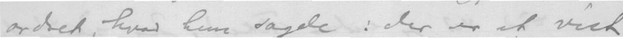ordet, hvad hun sagde: der er et vist
